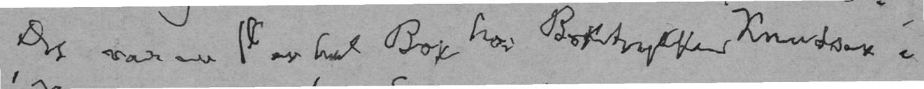Det var en | en hel Bok hos Boktrykker Knudsen i
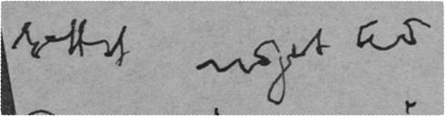rettet noget av
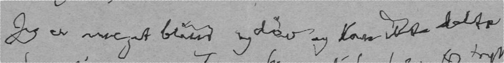Jeg er meget blind og døv og kan ikke delta
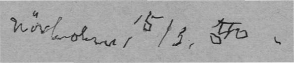Nørholm 15/6. 50.
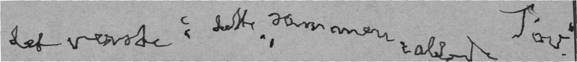det verste i dette "sammenrablede Tøv".
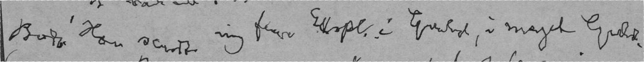Bodø. Han sendte mig flere Ekspl. i Guld, i meget Guld.
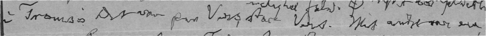i Tromsø. Det var paa Vers, store Vers. Mit andet var en
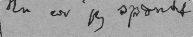Nu er jeg spændt
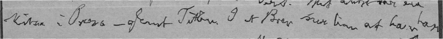Skitse i Prosa - glemt Titlen. I et Brev sier han at han har
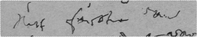Mitt første var
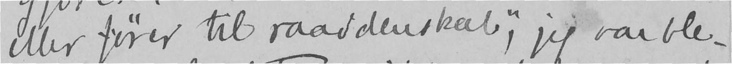eller fører til raaddenskab", jeg var ble-
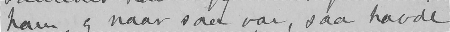ham, og naar saa var, saa havde
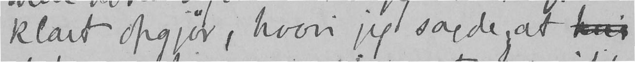klart opgjør, hvori jeg sagde, at hvis
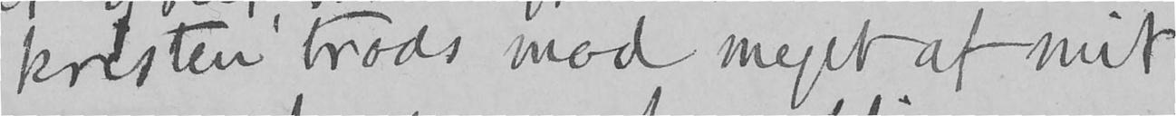kristen trods mod meget af mit,
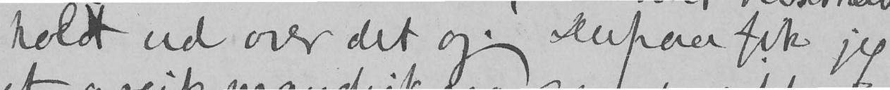holdt ud over det og. Derpaa fik jeg
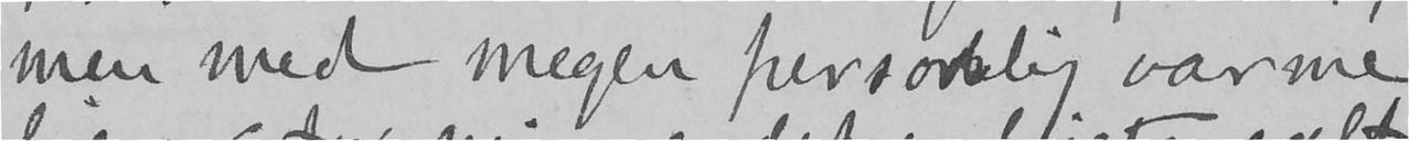men med megen personlig varme
